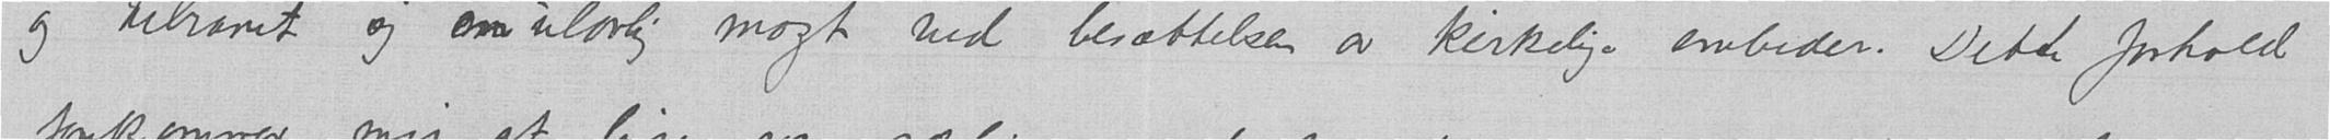og tilranet sig en ulovlig magt ved besættelsen av kirkelige embeder. Dette forhold
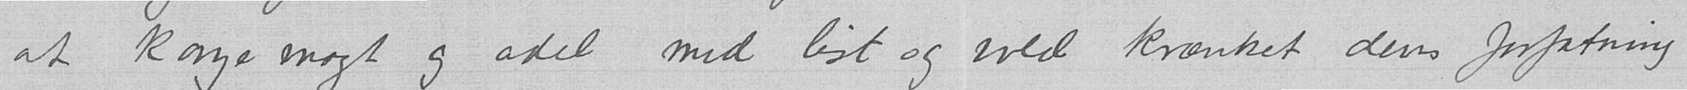at kongemagt og adel med list og vold krænket dens forfatning
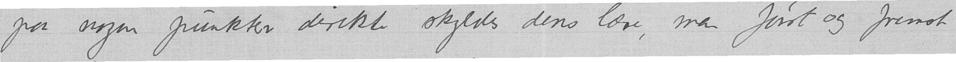paa nogen punkter direkte skyldes dens lære, men først og fremst
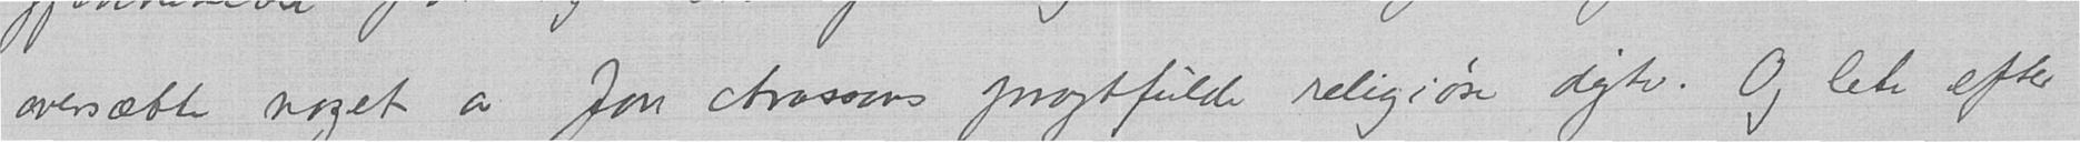oversætte noget av Jon Arassons pragtfulde religiøse digte. Og lete efter
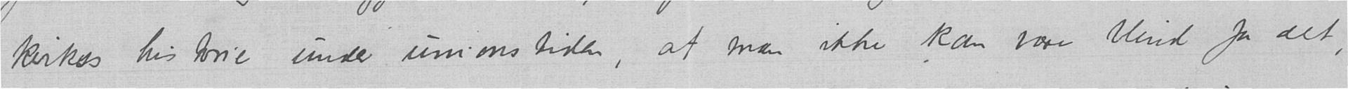kirkes historie under unionstiden, at man ikke kan være blind for det,
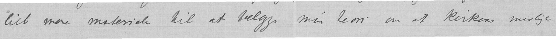litt mere materiale til at belegge min teori om at kirkens mislige
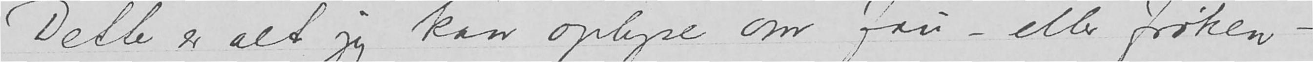Dette er alt jeg kan oplyse om fru – eller frøken –
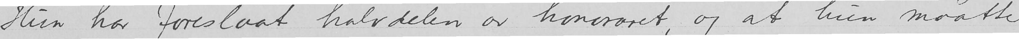Hun har foreslaat halvdelen av honoraret, og at hun maatte
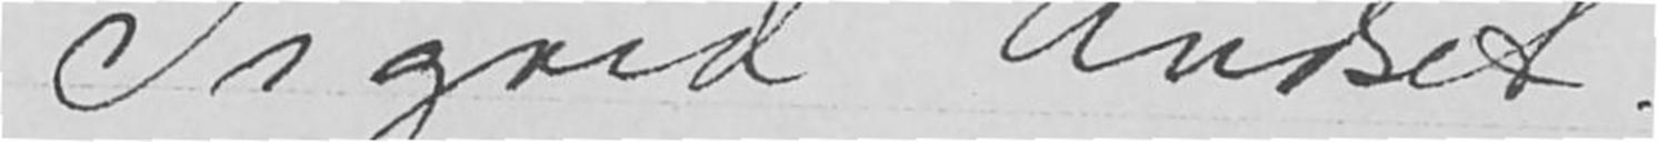Sigrid Undset.
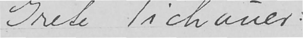Grete Tichauer:
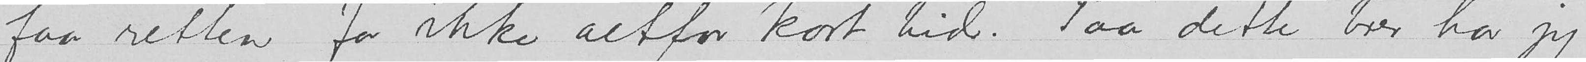faa retten for ikke altfor kort tid. Paa dette brev har jeg
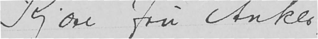Kjære Fru Anker,
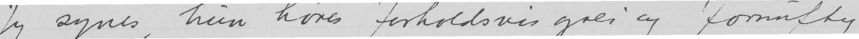Jeg synes, hun høres forholdsvis grei og fornuftig
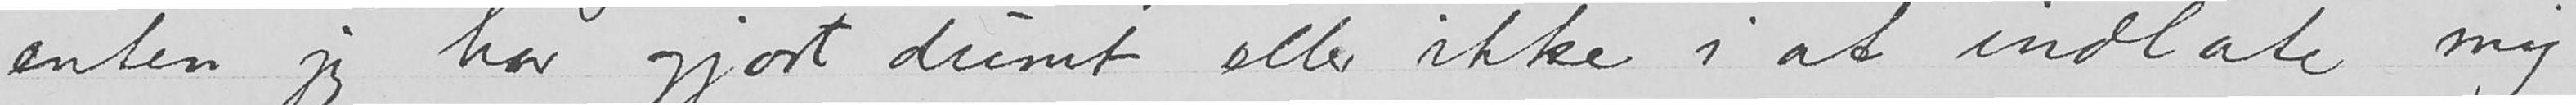enten jeg har gjort dumt eller ikke i at indlate mig
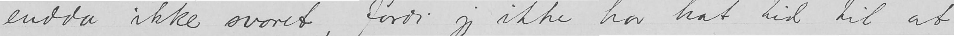endda ikke svaret, fordi jeg ikke har hat tid til at
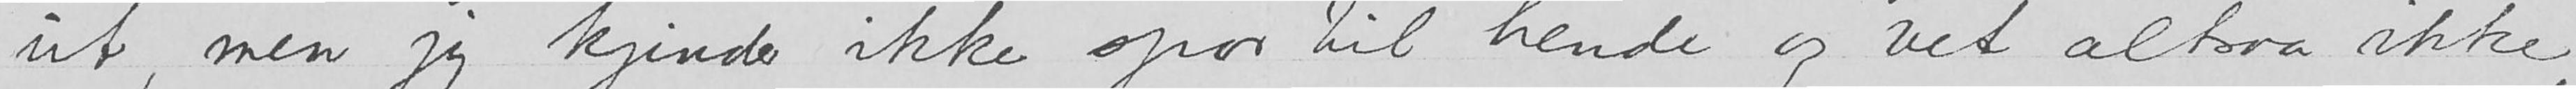ut, men jeg kjender ikke spor til hende og vet altsaa ikke
